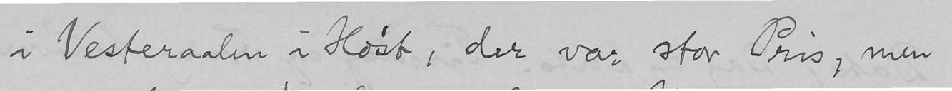i Vesteraalen i Høst, der var stor Pris, men
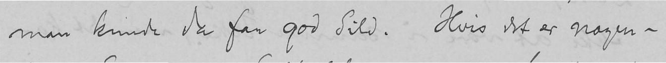man kunde da faa god Sild. Hvis det er nogen-
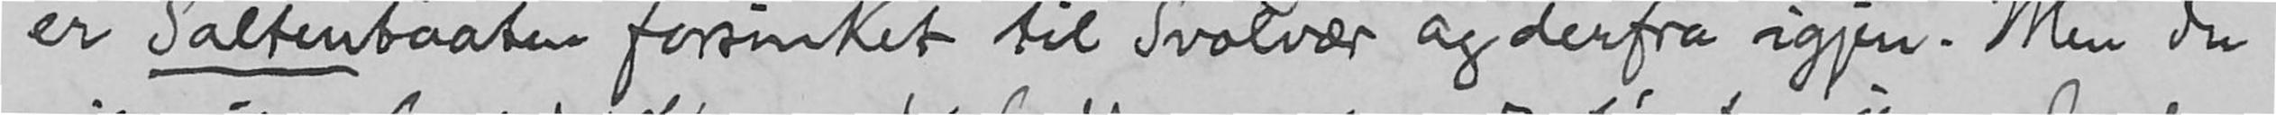er Saltenbaaten forsinket til Svolvær og derfra igjen. Men du
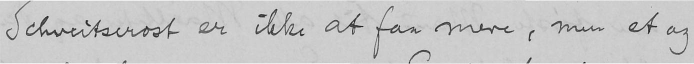Schveitserost er ikke at faa mere, men et og
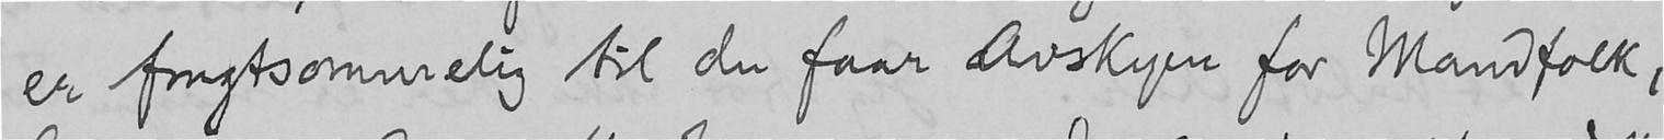er frugtsommelig til du faar Avskyen for Mandfolk,
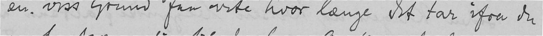en viss Grund faa vite hvor længe det tar ifra du
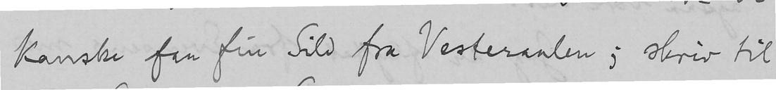kanske faa fin Sild fra Vesteraalen; skriv til
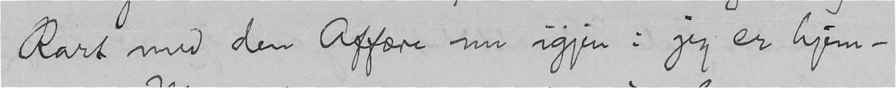Rart med den Affære nu igjen: jeg er hjem-
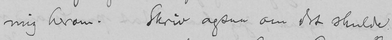mig herom. Skriv ogsaa om det skulde
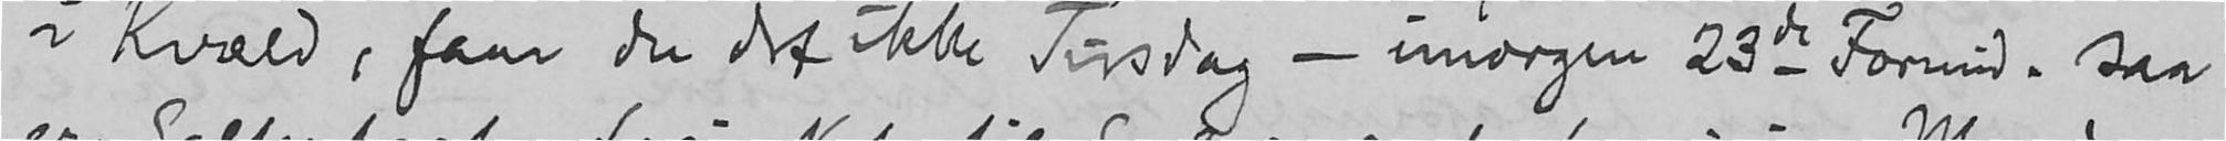i Kvæld, faar du det ikke Tirsdag - imorgen 23de Formid. saa
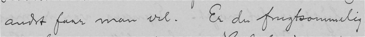andet faar man vel. Er du frugtsommelig
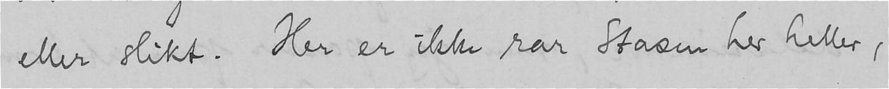eller slikt. Her er ikke rar Stasen her heller,
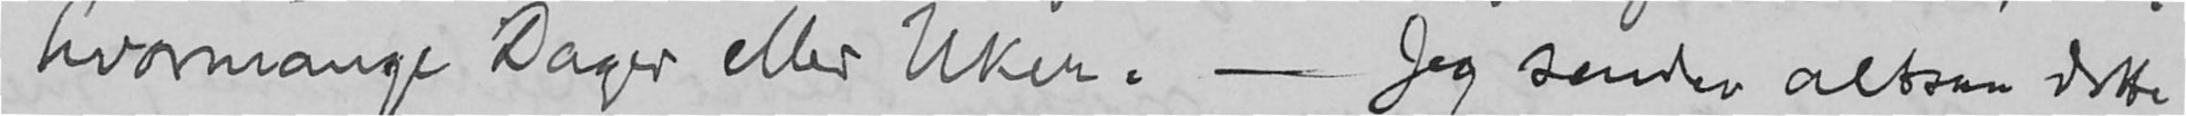hvormange Dager eller Uker. - Jeg sender altsaa dette
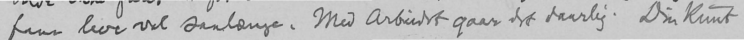faar leve vel saalænge. Med Arbeidet gaar det daarlig. Din Knut
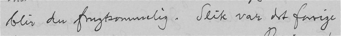blir du frugtsommelig. Slik var det forrige
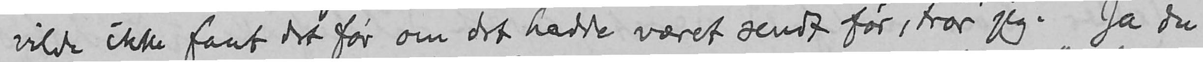vilde ikke faat det før om det hadde været sendt før, tror jeg. Ja du
